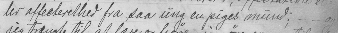ler affecterethed fra saa ung en piges mund; - - og
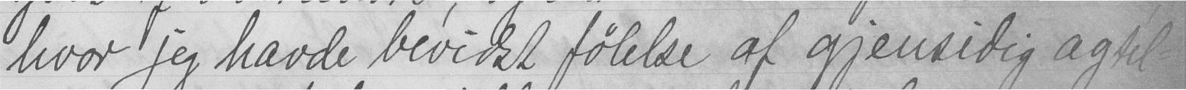hvor jeg havde bevidst følelse af gjensidig agtel-
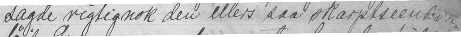sagde rigtignok den ellers saa skarptseende
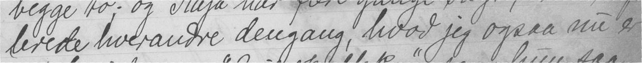lerede hverandre dengang, hvad jeg ogsaa nu er
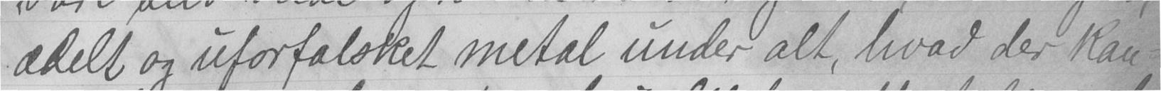ædelt og uforfalsket metal under alt, hvad der kan-
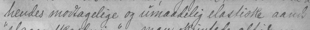hendes modtagelige og umaadelig elastiske aands
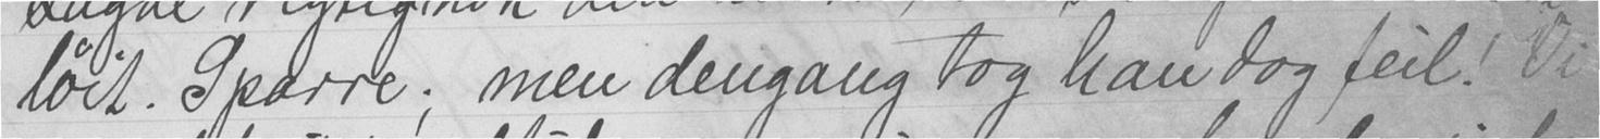løit. Sparre; men dengang tog han dog feil! Vi
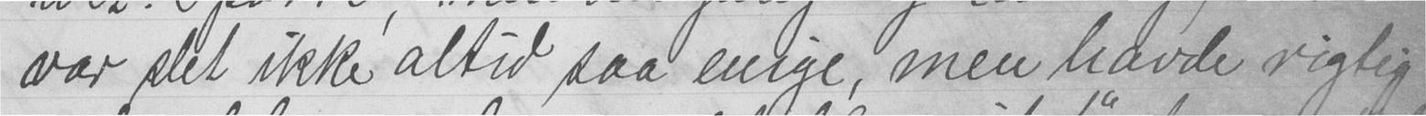var slet ikke altid saa enige, men havde rigtig
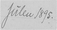Julen 1895.
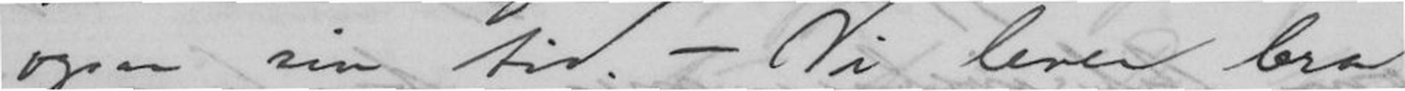ogsaa sin tid. - Vi lever bra
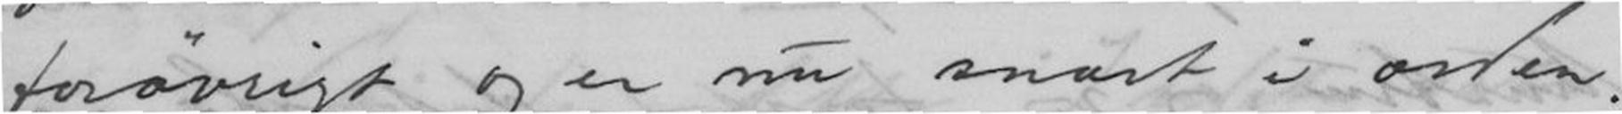forøvrigt og er nu snart i orden.
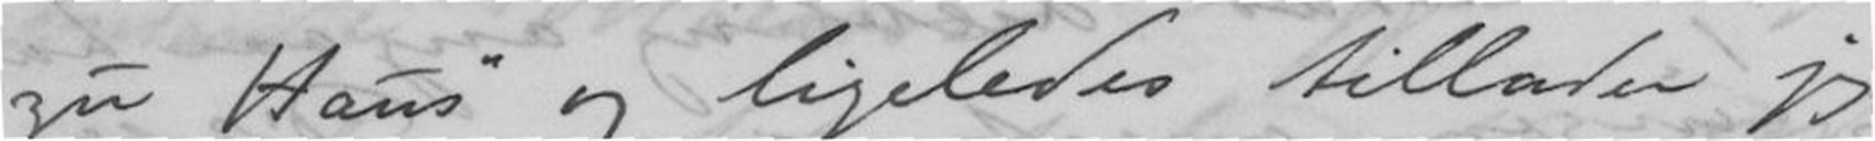zu Haus". og ligeledes tillader jeg
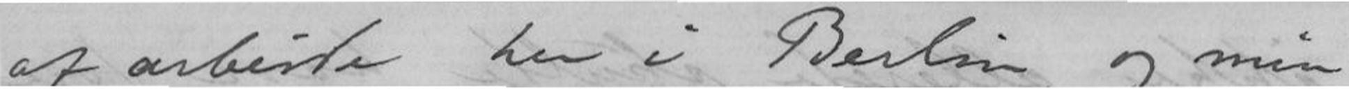af arbeide her i Berlin, og min
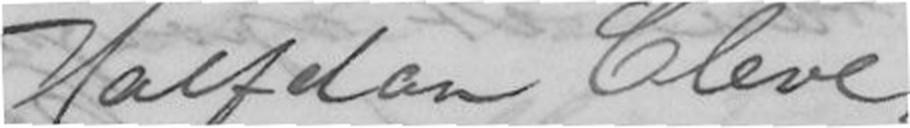Halfdan Cleve.
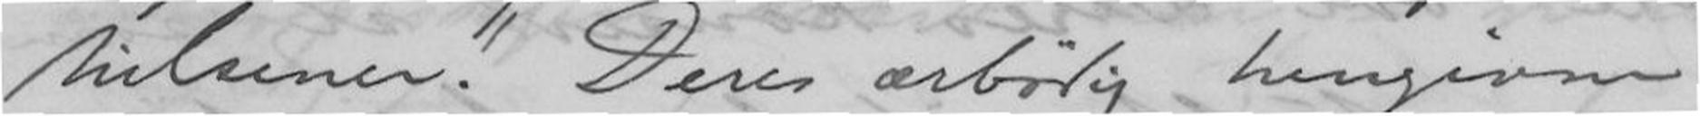hilsener! Deres ærbødig hengivne
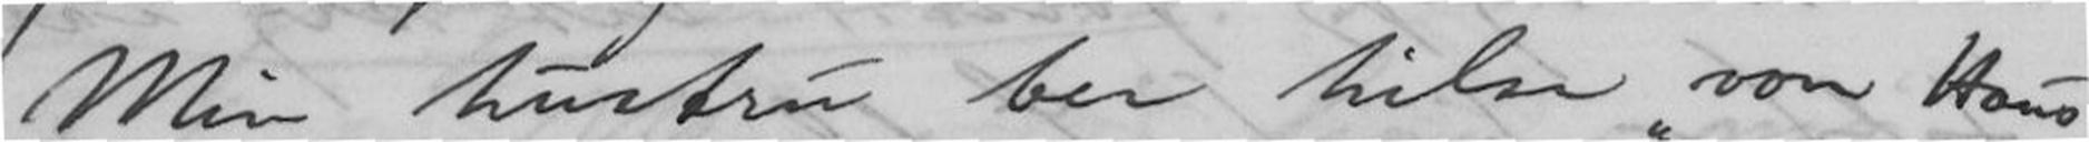Min hustru ber hilse "von Haus
